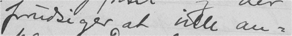forudsiger at ville an-
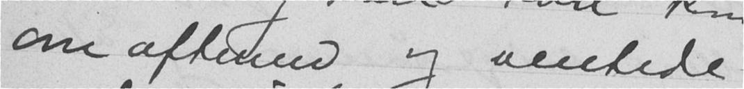om aftenen og ventede
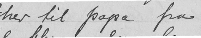brev til papa fra
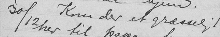30/12 Kom der et græsseligt
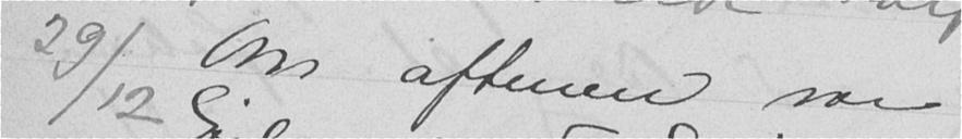29/12 Om aftenen var
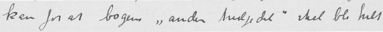kan for at bogens "anden tredjedel" skal bli helt
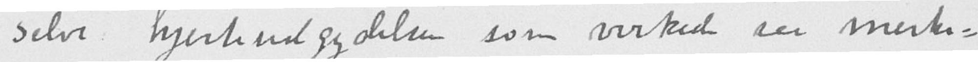selve hjerteudgydelsen som virkede saa merke-
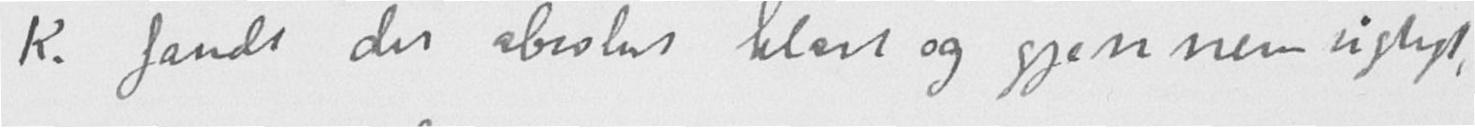K. fandt det absolut klart og gjennemsigtigt,
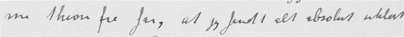me theon fra far, at jeg fandt alt absolut uklart
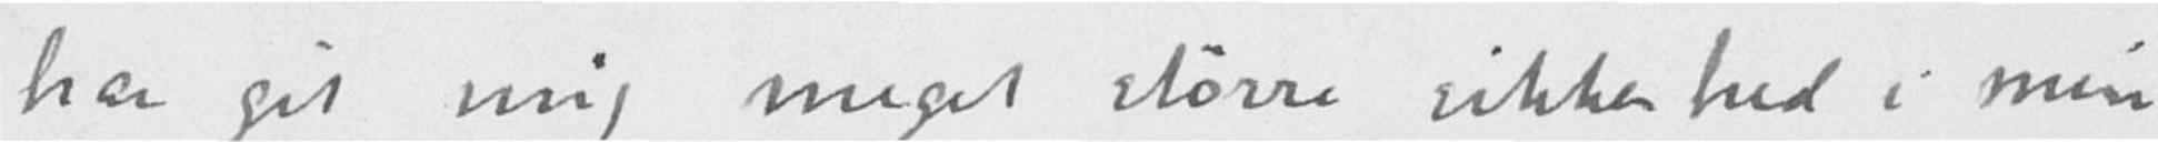har git mig meget større sikkerhed i min
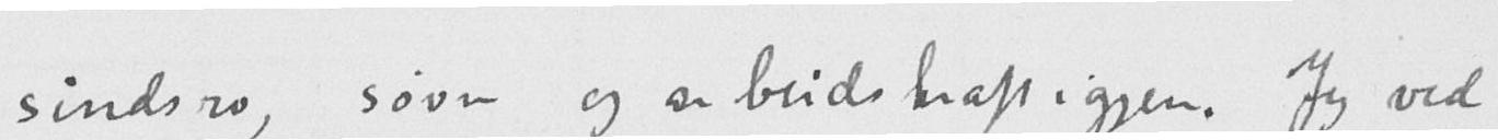sindsro, søvn og arbeidskraft igjen. Jeg ved
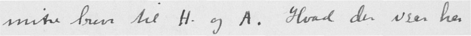mine breve til H. og A. Hvad der især har
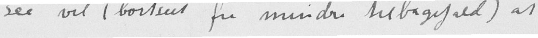saa vel (bortseet fra mindre tilbagefald) at
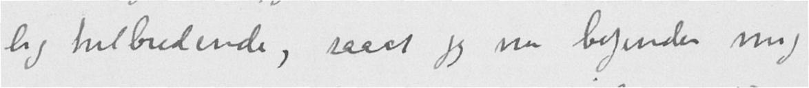lig helbredende, saaat jeg nu befinder mig
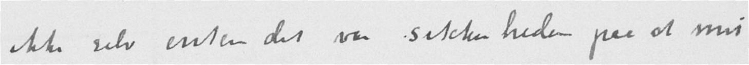ikke selv enten det var sikkerheden paa at mit
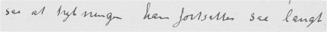saa at trykningen kan fortsætte saa langt
